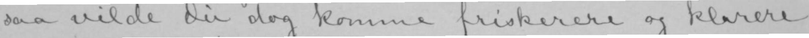saa vilde Du dog komme friskerere og klarere
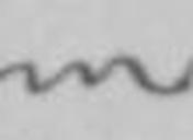om
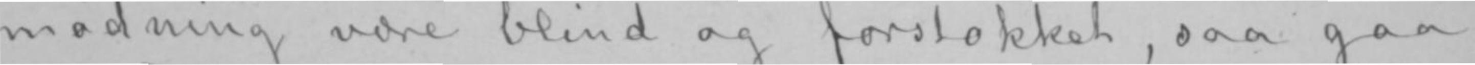modning være blind og forstokket, saa gaa
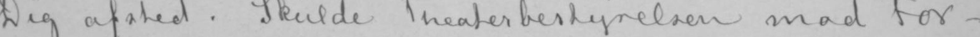Dig afsted. Skulde Theaterbestyrelsen mod For-
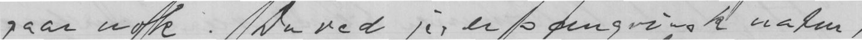gaar nok. Du ved jeg er sangvinsk natur,
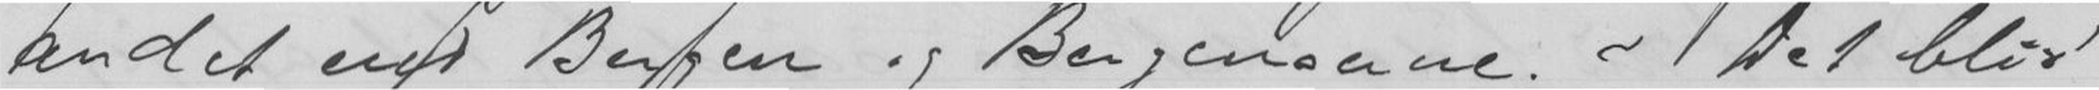andet end i Bergen og Bergenserne. - Det blir
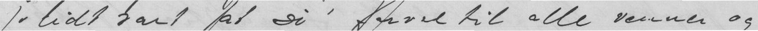jo lidt rart at si farvel til alle venner og
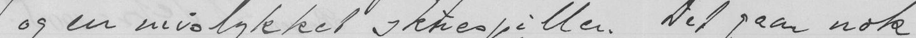og en mislykket skuespiller. Det gaar nok
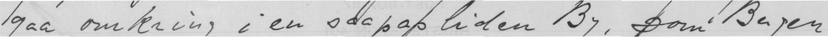gaa omkring i en saapas liden By, som Bergen,
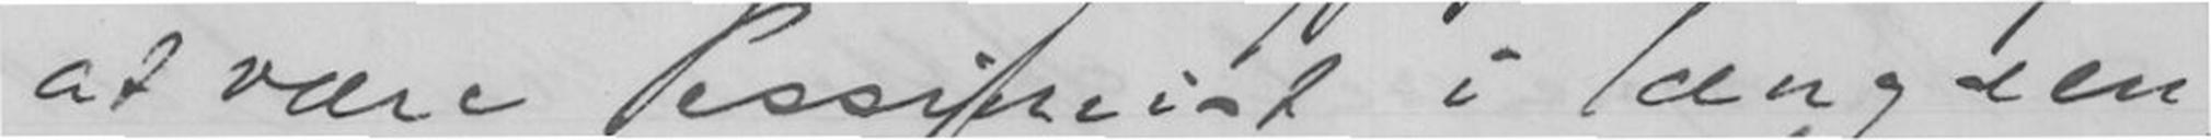at være Pessimist i længden.
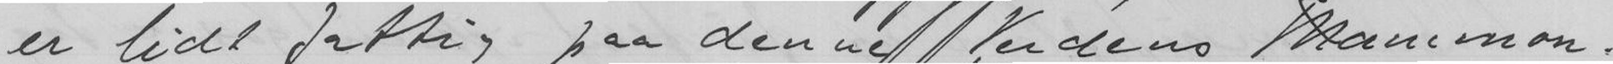er lidt fattig paa denne Verdens Mammon.
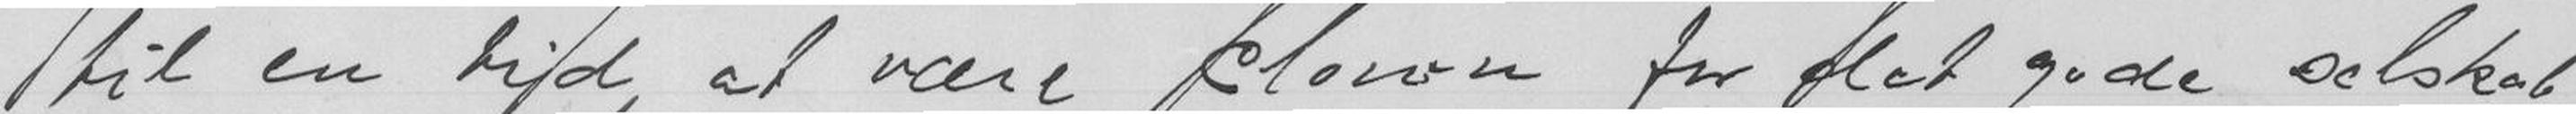til en tid at være clovn for det gode selskab
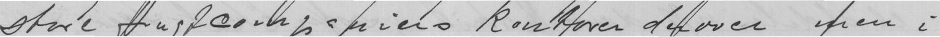store frugtcompaniers kontorer derover, men i
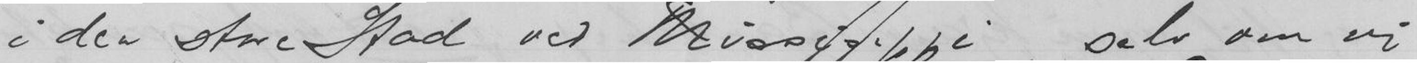i den store Stad ved Missisippi, selv om vi
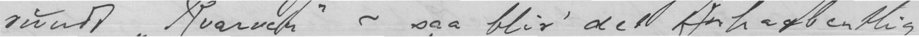rundt Kvarven - saa blir' det forhaabentlig
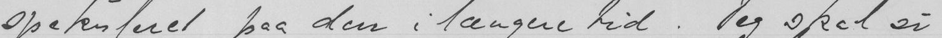spekuleret paa den i længere tid. Jeg skal si'
